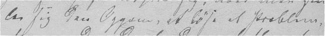ler sig den Opgave, at løse et Problem,
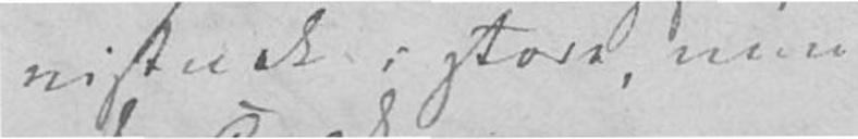vistnok i store, men
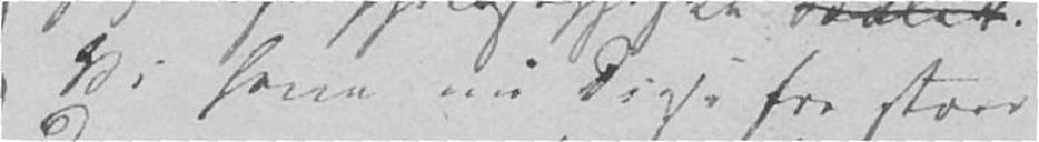Vi have nu disse tre store
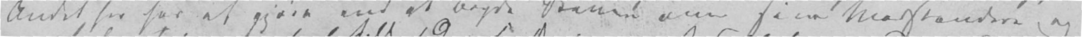Andet her har at gjøre end at bryde Staven over sine Modstandere og
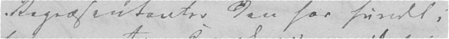Repræsentanter den har fundet i
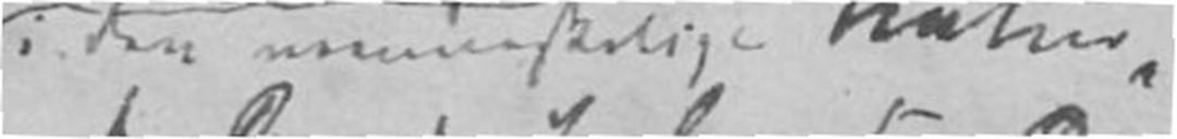i den menneskelige Natur,
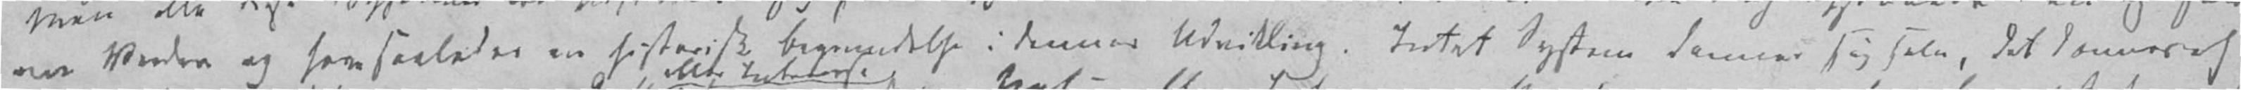me Verden og have saaledes en historisk Begyndelse i dennes Udvikling. Intet System danner sig selv, det dannes af
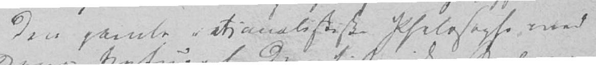Den gamle retsanalytiske Philosophe med
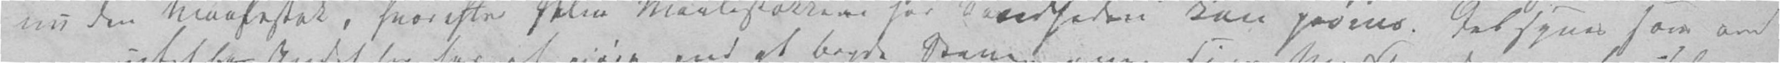nu den Maalestok, hvorefter selve Maalestokken for Sandheden kan prøves. Det synes som om
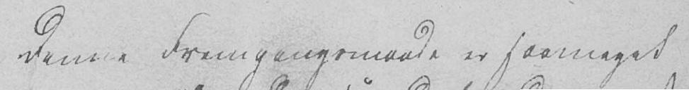Denne Fremgangsmaade er saameget
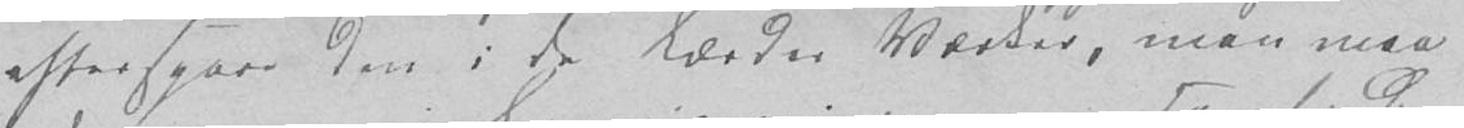efterspore den i de lærde Værker, man maa
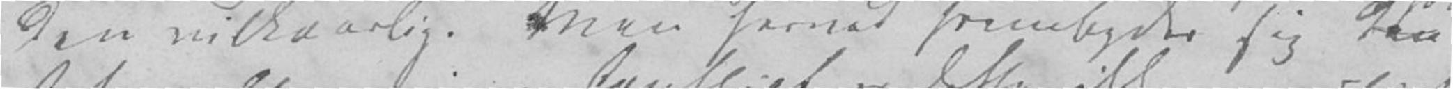den vilkaarlig. Men herved frembyder sig den
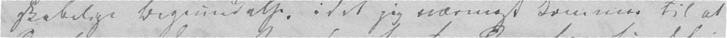skabelige Begrundelse, idet jeg nærmest kommer til at
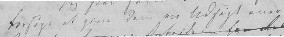forsøge at give Dem en Udsigt over
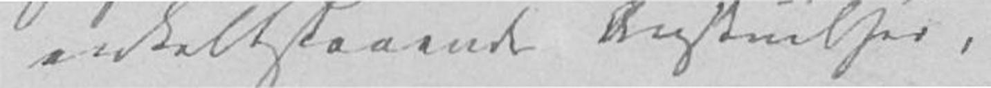enkeltstaaende Anskuelser,
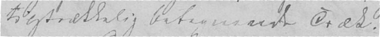tilstrækkelig betegnende Træk.
